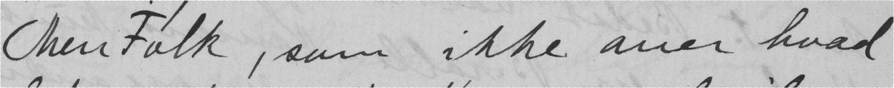Men Folk, som ikke aner hvad
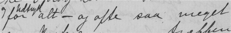for alt - og ofte saa meget
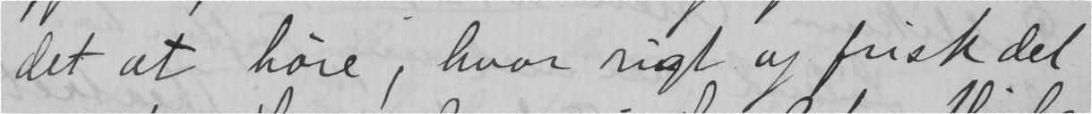det at høre, hvor rigt og frisk det
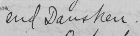end Dansken.
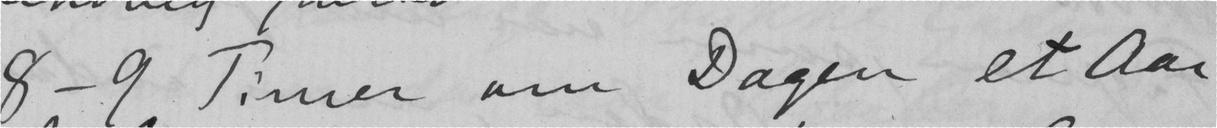8 - 9 Timer om Dagen et Aar
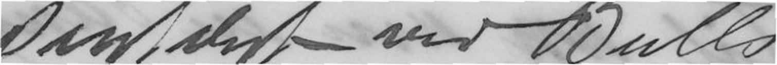sentæret ved Bulls
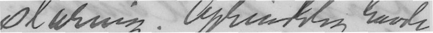sløring. Oprindelig havde
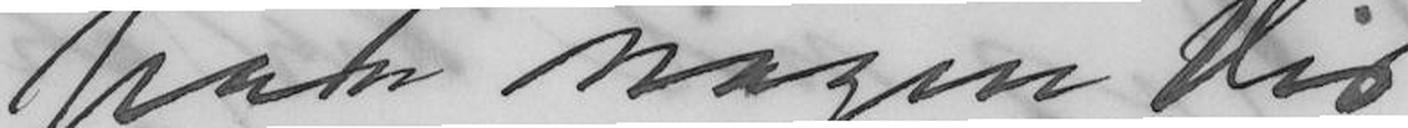paa nogen Vis
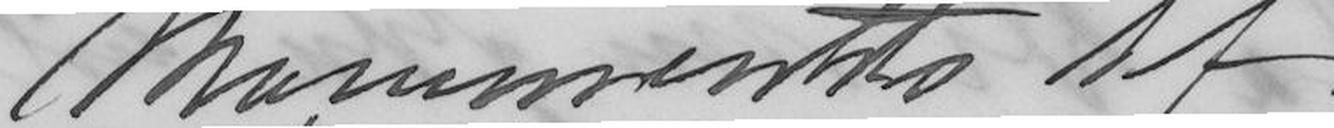Monumentets Af-
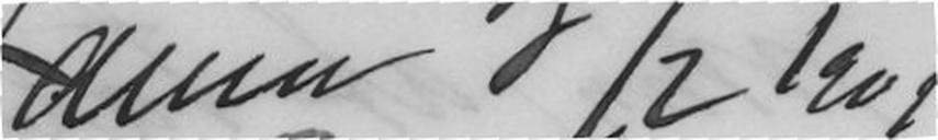Xania 5/2 1901
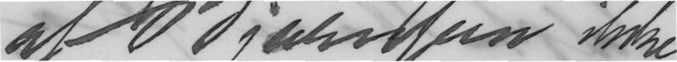at Bjørnson ikke
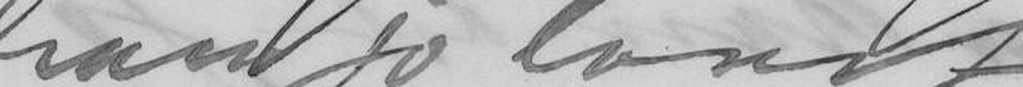han jo lovet
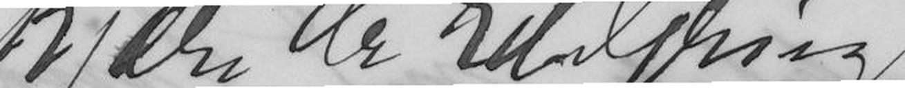Kjære Hr Edv Grieg
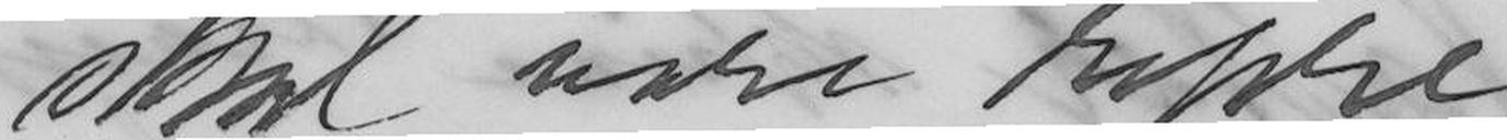skal være repre-
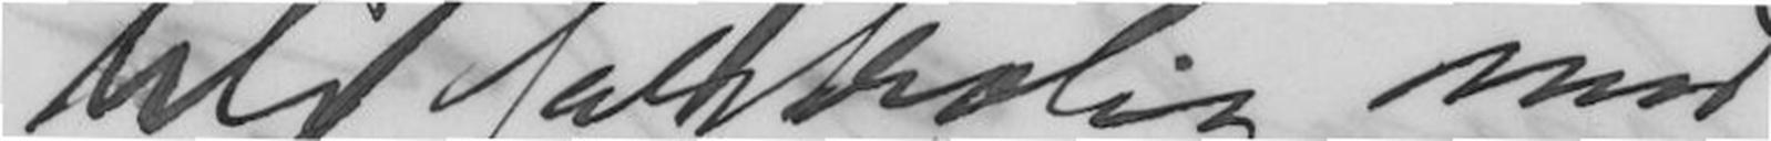bli fortrolig med
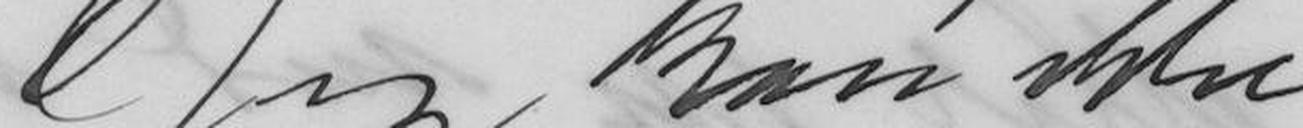Jeg kan ikke
